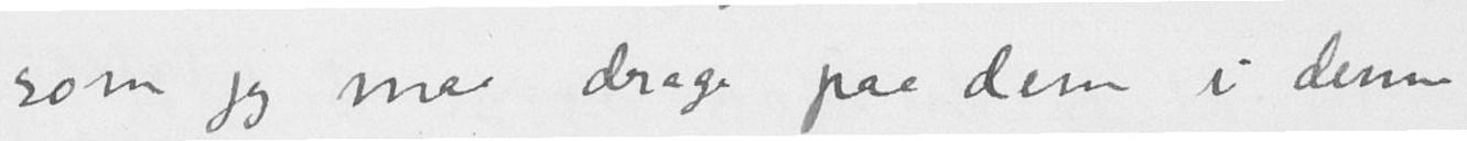som jeg maa drage paa dem i denne
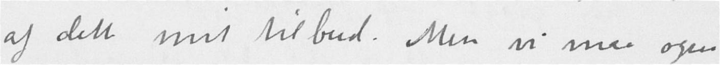af dette mit tilbud. Men vi maa ogsaa
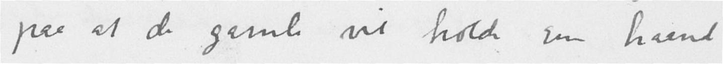paa at de gamle vil holde sin haand
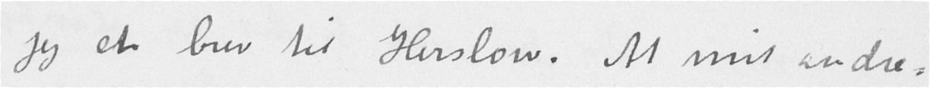jeg et brev til Herslow. At mit andra-
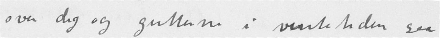over dig og gutterne i ventetiden saa
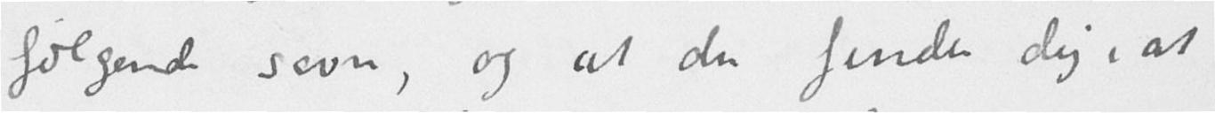følgende savn, og at du finder dig i at
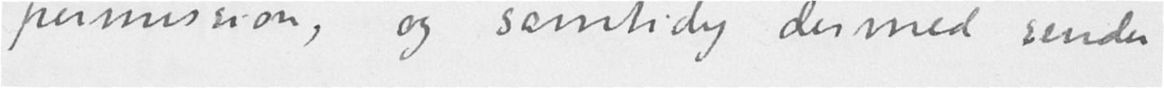permission, og samtidig dermed sender
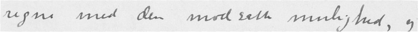regne med den modsatte mulighed, og
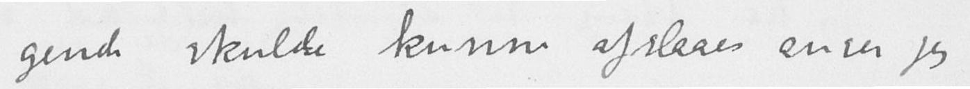gende skulde kunne afslaaes anser jeg
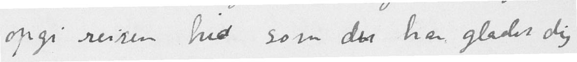opgi reisen hid som du har glædet dig
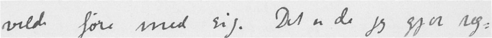vilde føre med sig. Det er da jeg gjør reg-
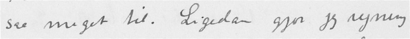saa meget til. Ligedan gjør jeg regning
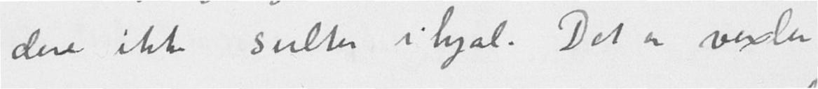dere ikke sulter i hjæl. Det er vexler
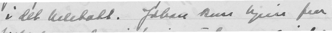i det heletatt. Johan kom hjem fra
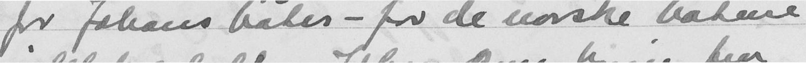for Johans båter - for de norske båtene
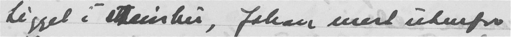Ligget i steinhus, Johan mest utenfor
